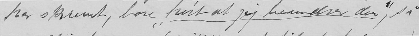har skrevet, bare "hørt at jeg beundrer den", så
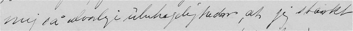mig så alvorlig i ubehageligheder, at jeg stærkt
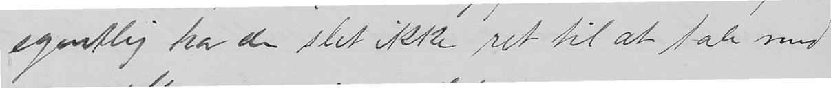egentlig har de slet ikke ret til at tale med
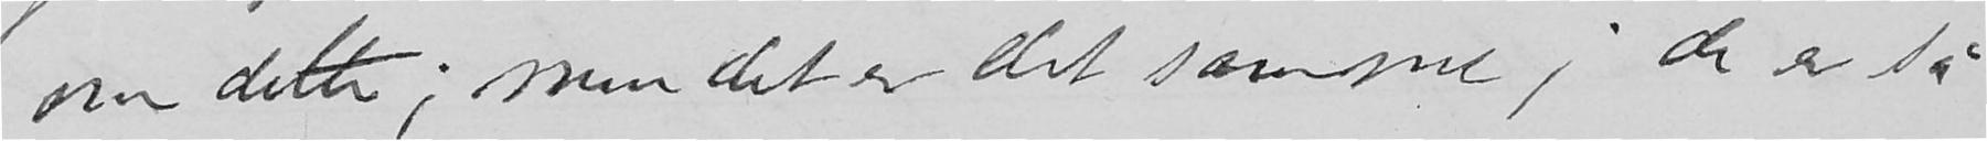om dette; men det er det samme; de er så
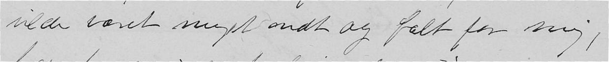vilde været meget ondt og fælt for mig,
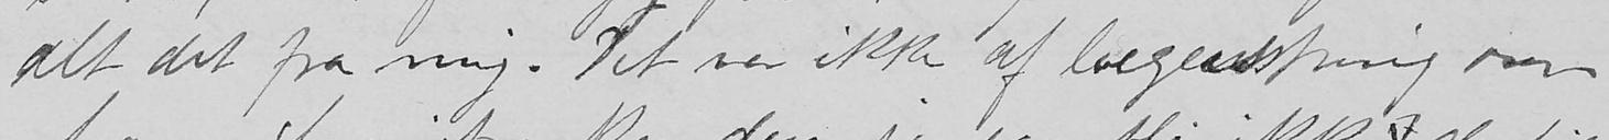alt det fra mig. Det var ikke af begeistring over
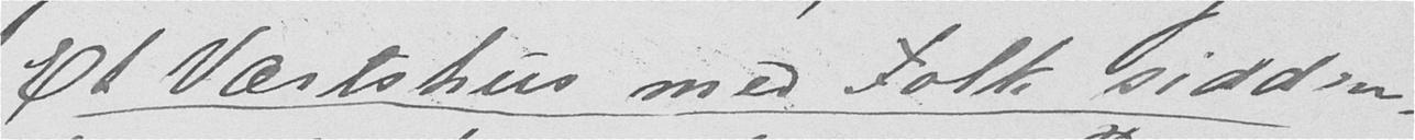Et Værtshus med Folk sidden-
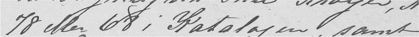78 eller 68 i Katalogen, samt
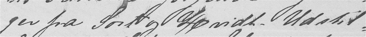ger fra Sort og Hvidt-Udstil-
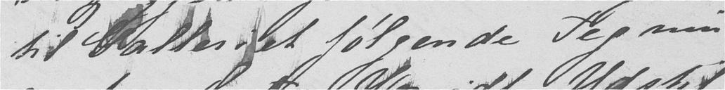til Galleriet følgende Tegnin-
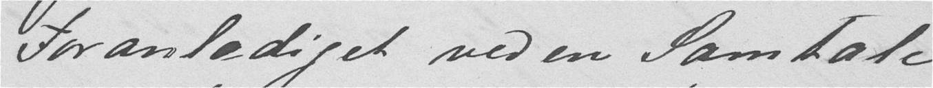Foranlediget ved en Samtale
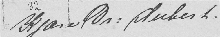Kjære Dr. Aubert.
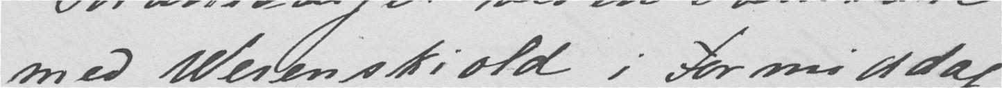med Werenskiold i Formiddag,
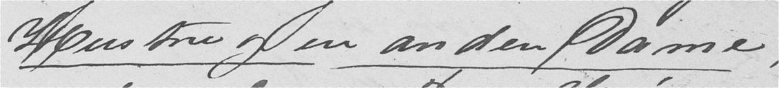Hustru og en anden Dame,
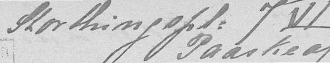Storthingspl. 7 Vl
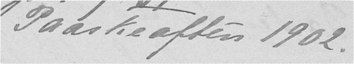Paaskeaften 1902.
